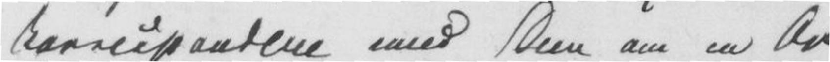korrespondere med Dem om en Or-
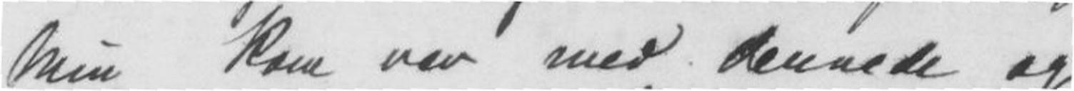Min kone var med dernede og
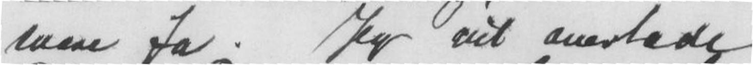svare Ja. Jeg vil overlade
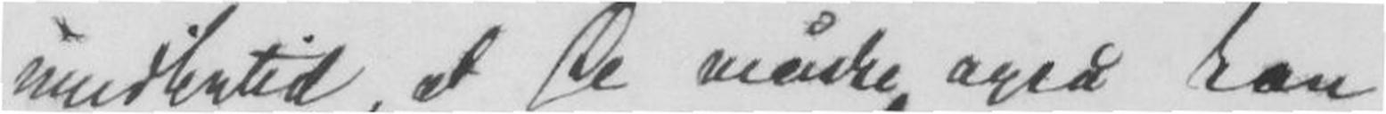imidlertid, at De måcke også kan
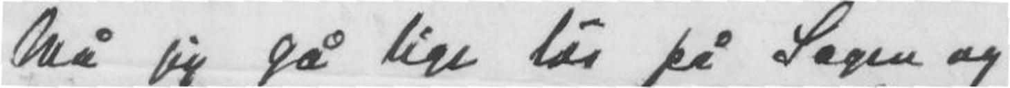Må jeg gå lige løs på Sagen og
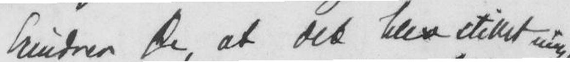Erindrer De, at det hendstillet -
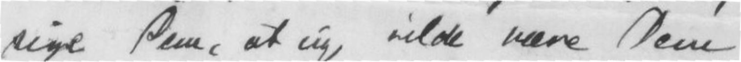sige Dem, at jeg vilde være Dem
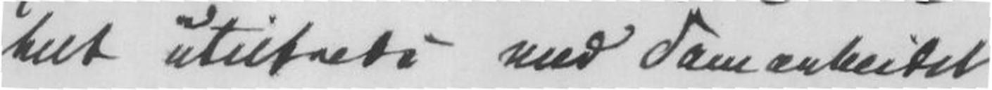utilfreds med Samanheider
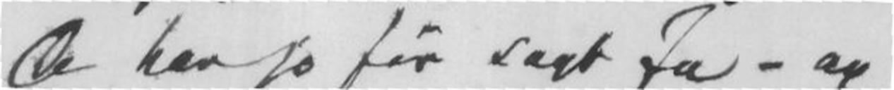De har jo før sagt ja- og
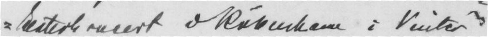kesterkoncert i Köbenhavn i Vinter
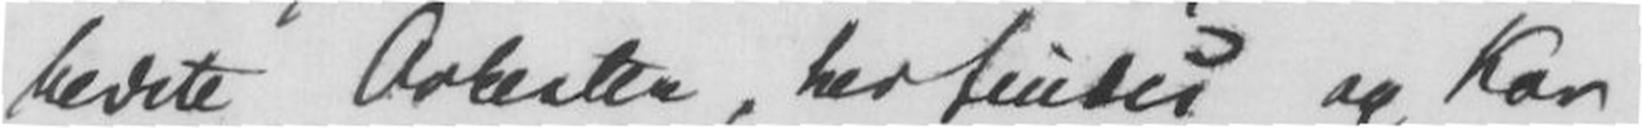bedste Orkester, her findes og kan
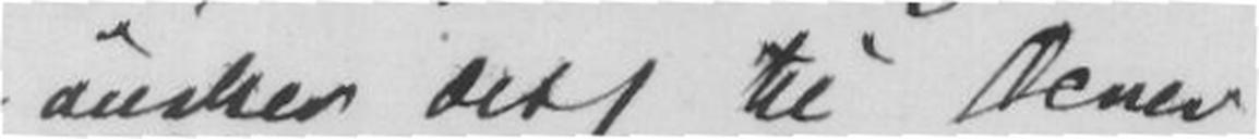ønsker det, til Deres
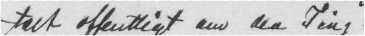talt offentligt om den Ting.
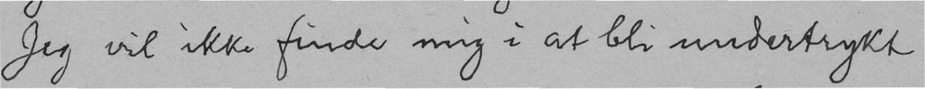Jeg vil ikke finde mig i at bli undertrykt
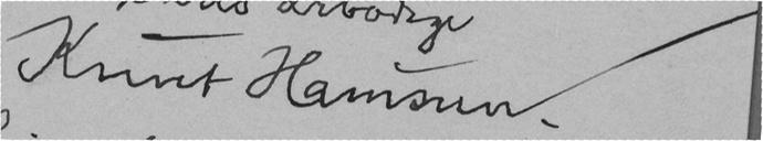Knut Hamsun.
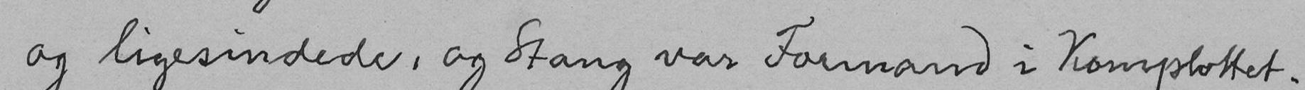og ligesindede, og Stang var Formand i Komplottet.
